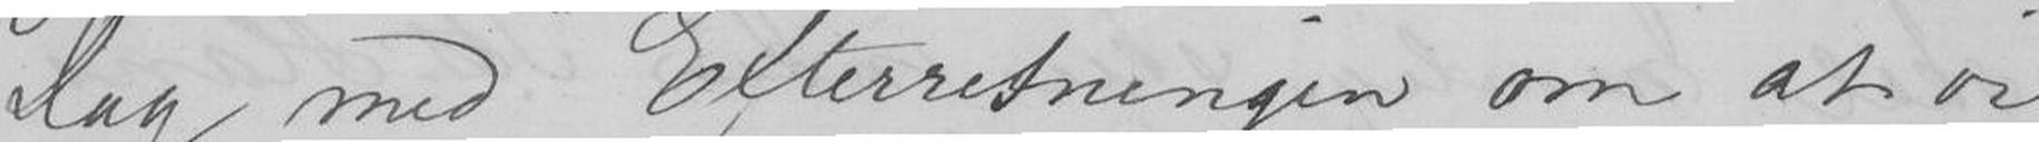Dag med Efterretningen om at vi
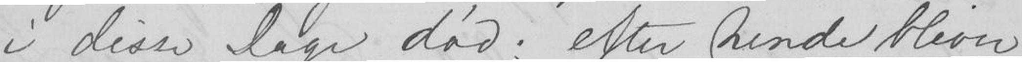i disse Dage død; efter hende bliver
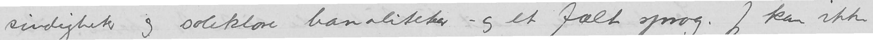sindigheter og soleklare banaliteter – og et fælt sprog. Jeg kan ikke
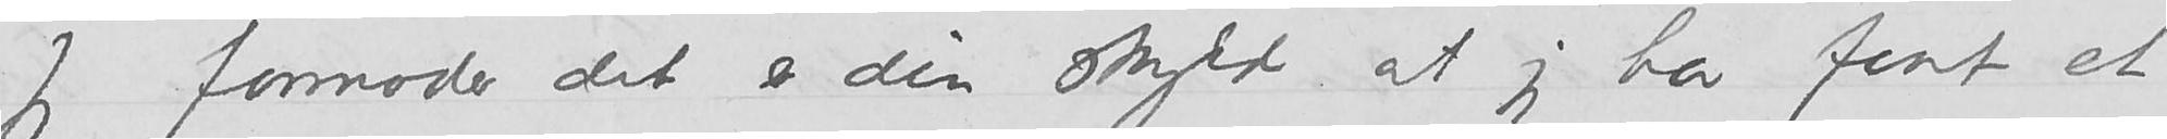Jeg formoder det er din skyld at jeg har faat et
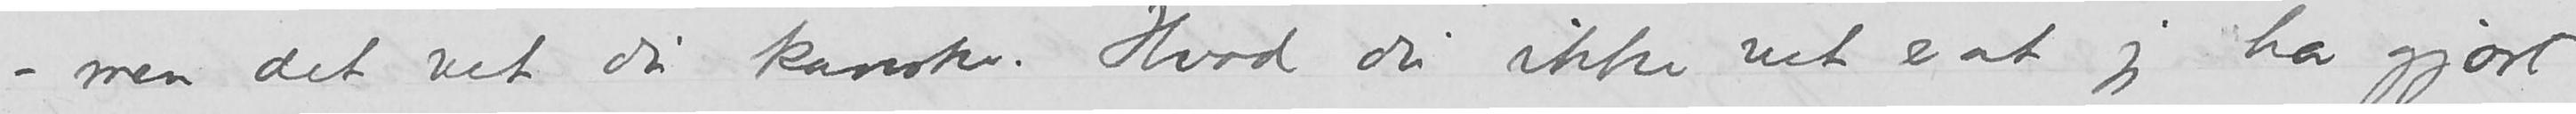– men det vet du kanske. Hvad du ikke vet er at jeg har gjort
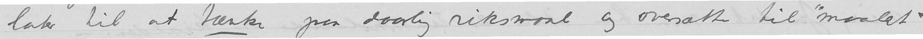later til at tænke paa daarlig riksmaal og oversætter til «maalet»
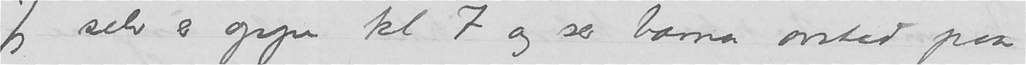Jeg selv er oppe kl 7 og ser barna avsted paa
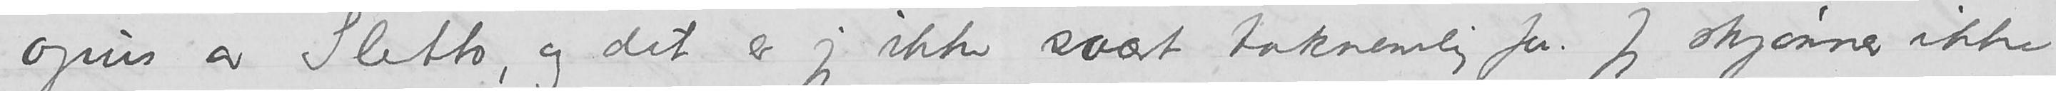opus av Sletto, og det er jeg ikke svært taknemlig for. Jeg skjønner ikke
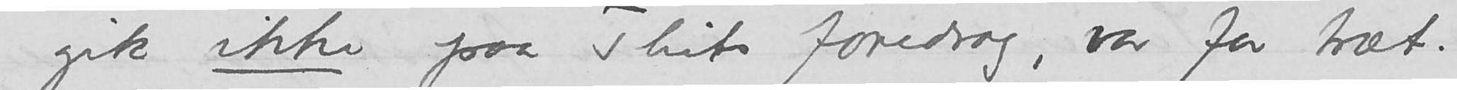Jeg gik ikke paa Thits foredrag, var for træt.
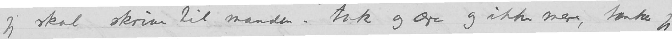jeg skal skrive til manden – tak og ære og ikke mere, tænker jeg.
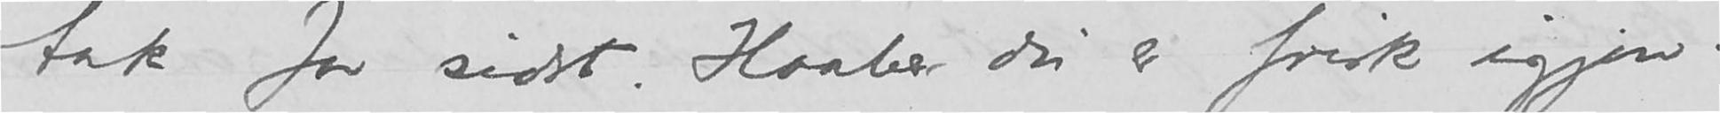tak for sidst. Haaber du er frisk igjen.
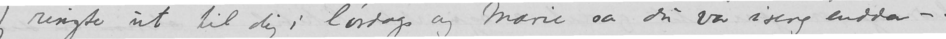Jeg ringte ut til dig i lørdags og Marie sa du var iseng endda –.
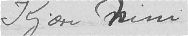Kjære Nini
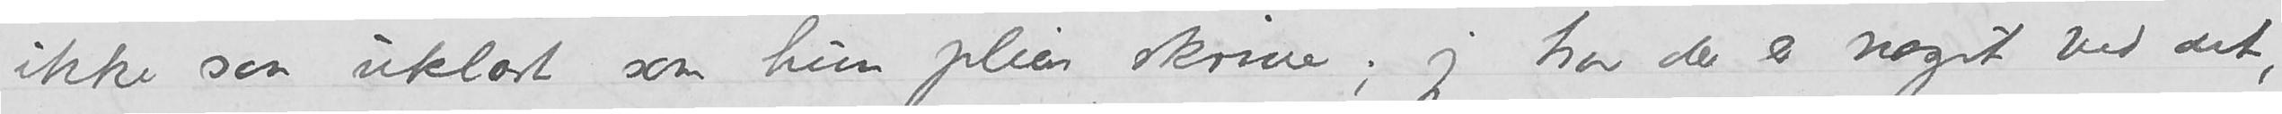ikke saa uklart som hun pleier skrive; jeg tror der er noget ved det,
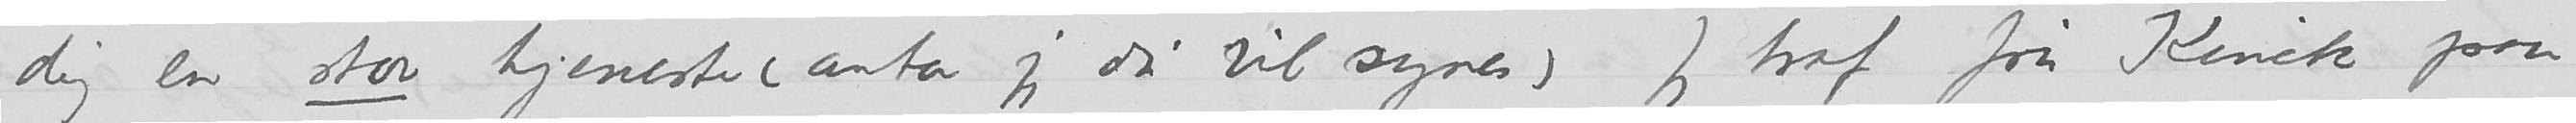dig en stor tjeneste (antar jeg du vil synes) Jeg traf fru Kinck paa
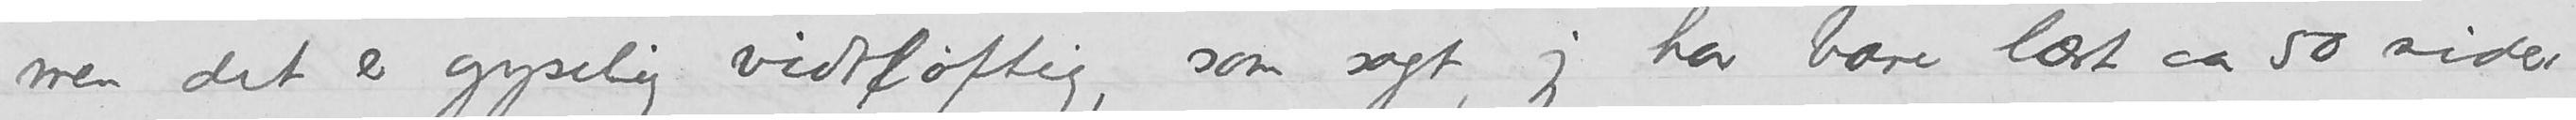men det er gyselig vidtløftig, som sagt, jeg har bare læst ca 50 sider
